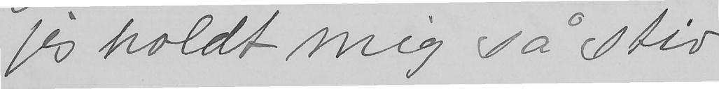jeg holdt mig så stiv
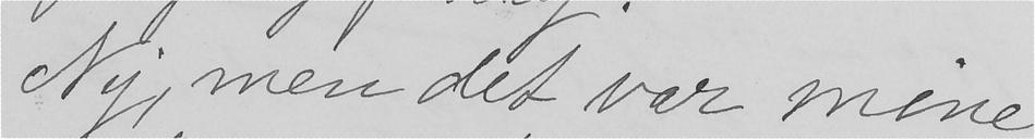Nej, men det var mine
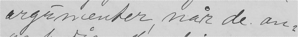argumenter, når de an-
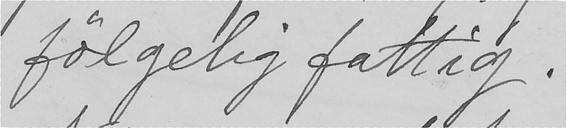følgelig fattig.
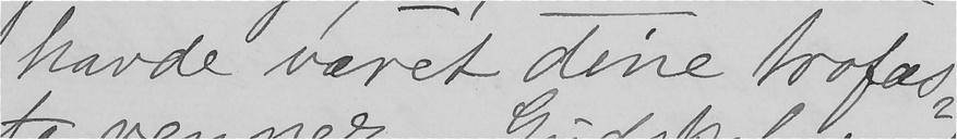havde været dine trofas-
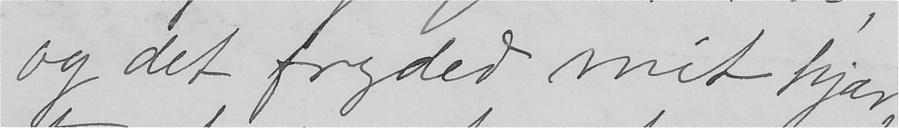og det fryded mit hjær-
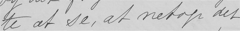te at se, at netop det
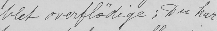blet overflødige: Du har
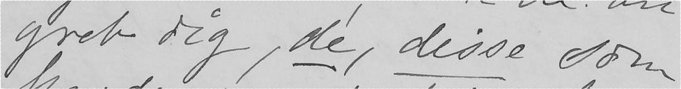greb dig, de, disse som
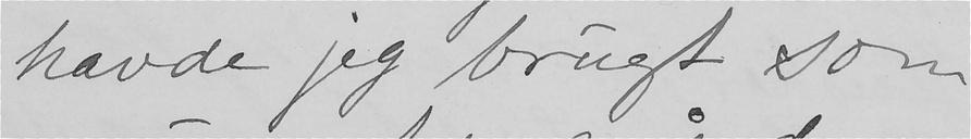havde jeg brugt som
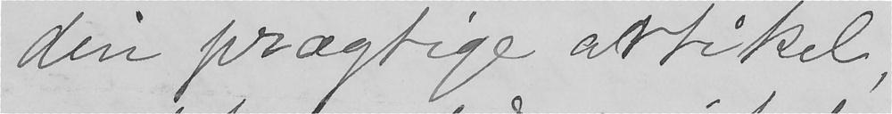din prægtige artikel,
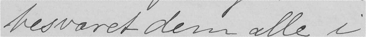besvaret dem alle i
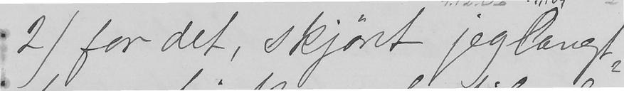2) for det, skjønt jeg langt-
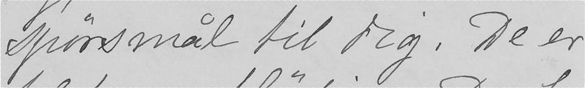spørsmål til dig. De er
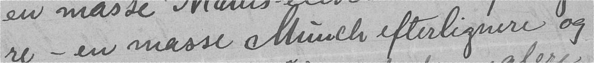re - en masse Munch efterlignere og
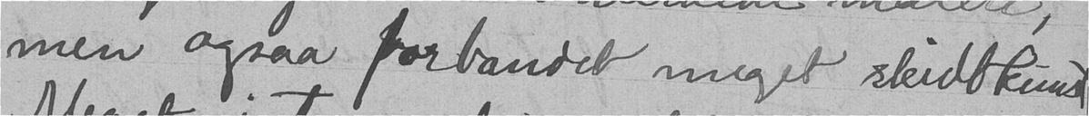men ogsaa forbandet meget skidtkunst.
